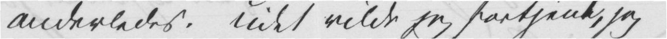anderledes. Lidet vilde jeg fortjent, jeg
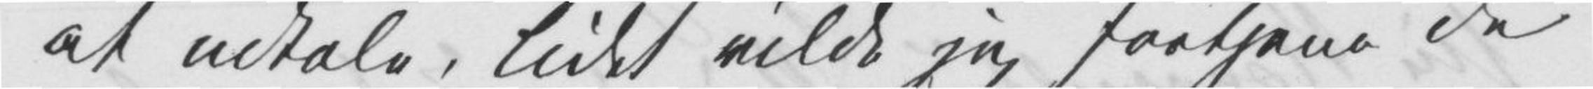at udtale, lidet vilde jeg fortjene de
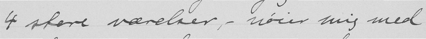4 store værelser, - nøier mig med
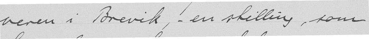veren i Brevik, - en stilling, som
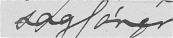sagfører
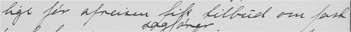lige før afreisen fik tilbud om fast
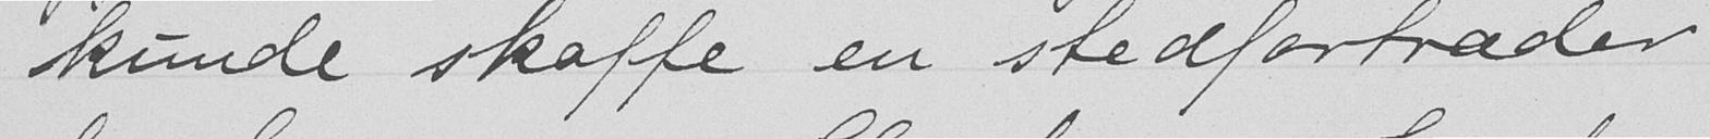kunde skaffe en stedfortræder
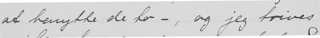at benytte de to -, og jeg trives
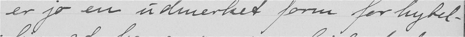er jo en udmerket form for hybel-
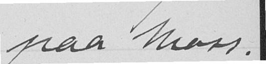paa Moss.
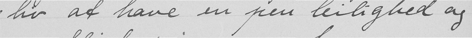liv at have en pen leilighed og
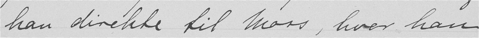han direkte til Moss, hvor han
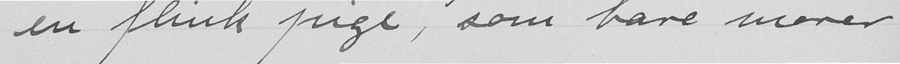en flink pige, som bare morer
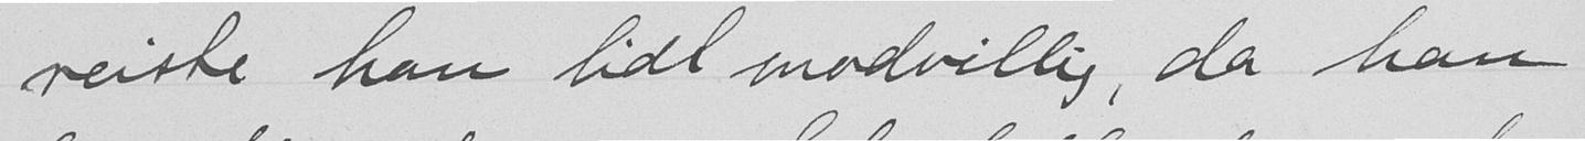reiste han lidt modvillig, da han
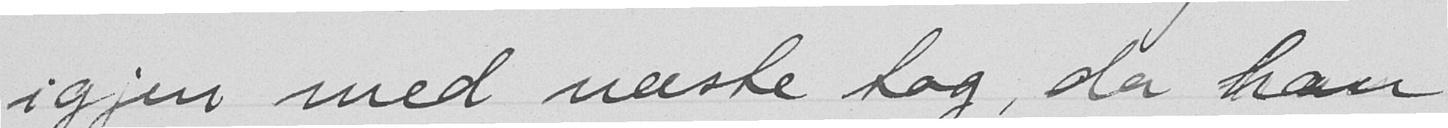igjen med næste tog, da han
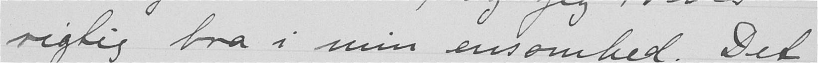rigtig bra i min ensomhed. Det
